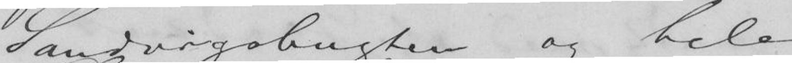Sandvigsbugten og hele
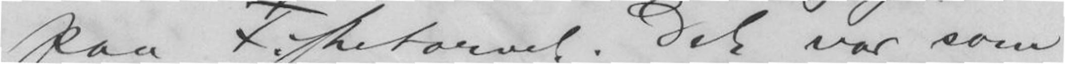paa Fisketorvet. Det var som
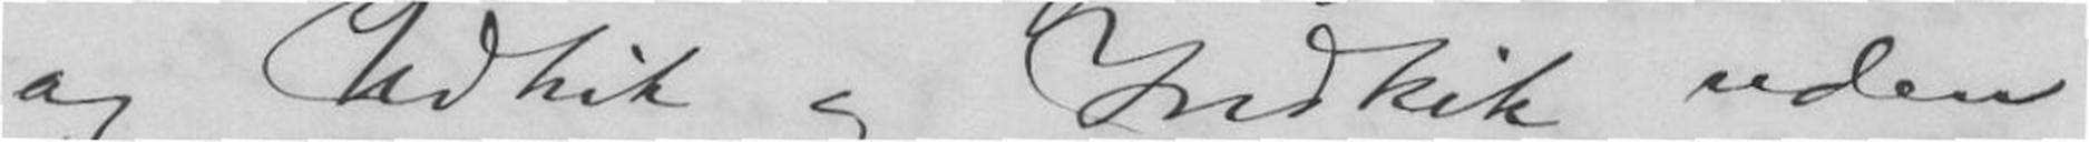og Udkik og Indkik uden
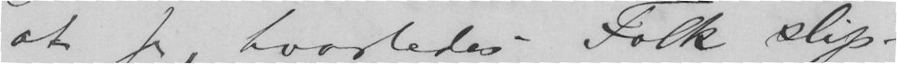at se, hvorledes Folk slip-
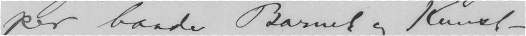per baade Barnet og Kunst-
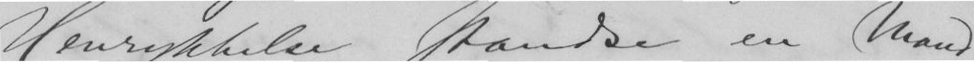Henrykkelse standse en Mand,
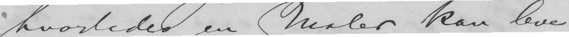hvorledes en Maler kan leve
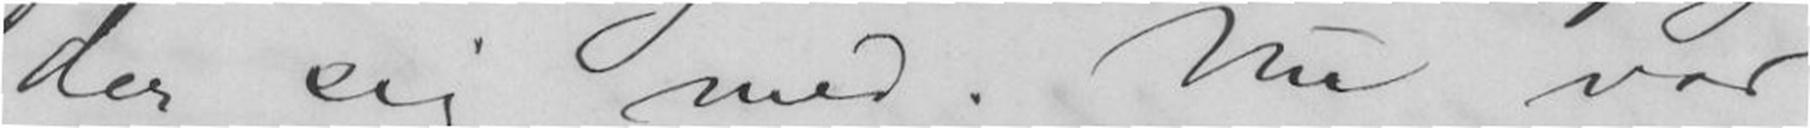der sig med. Nu var
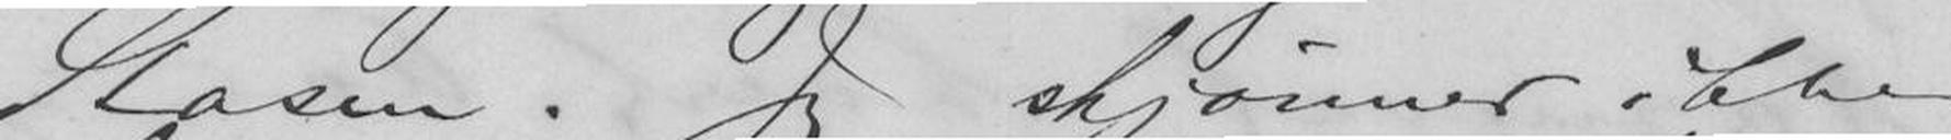Stasen. Jeg skjønner ikke,
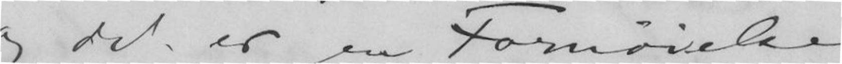og det er en Fornøielse
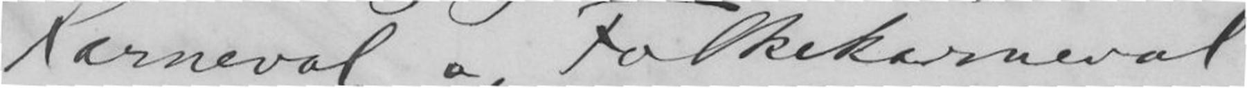Karneval og Folkekarneval
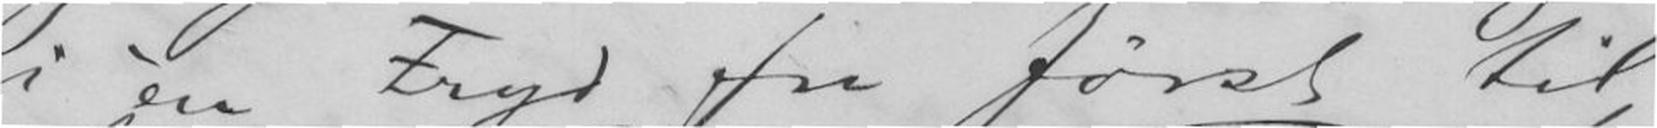i en Fryd fra først til
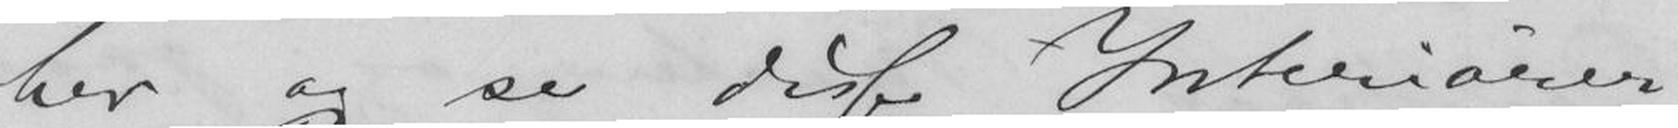her og se disse Interiører
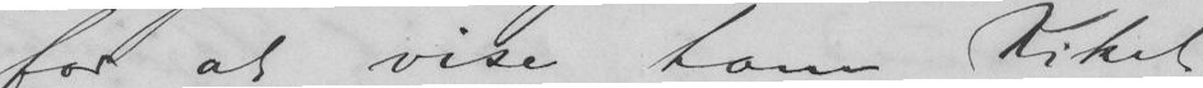for at vise ham Kiket
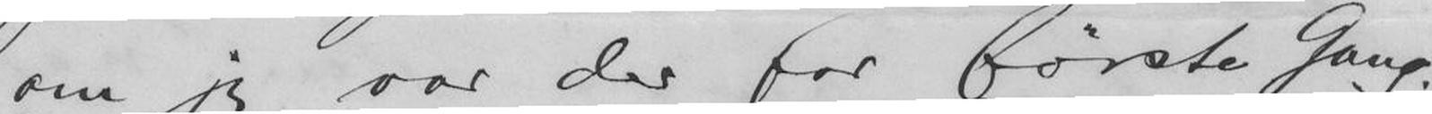om jeg var der for første Gang.
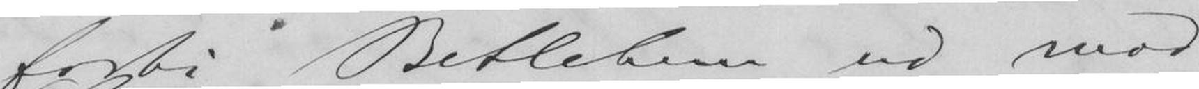forbi Betlehem ud mod
